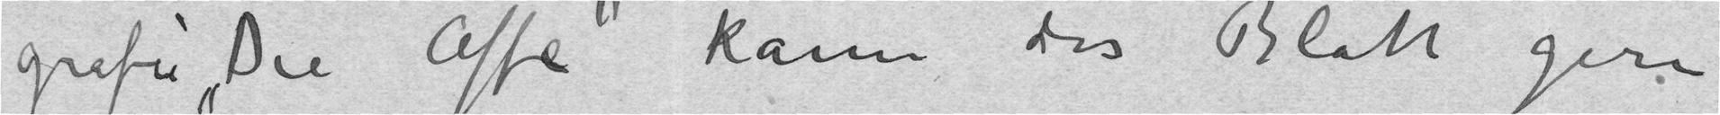grafie «Die Affe» kann das Blatt gern
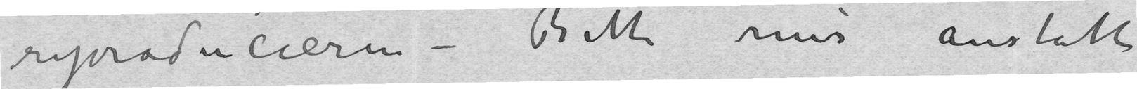reproducieren – Bitte mir anstatt
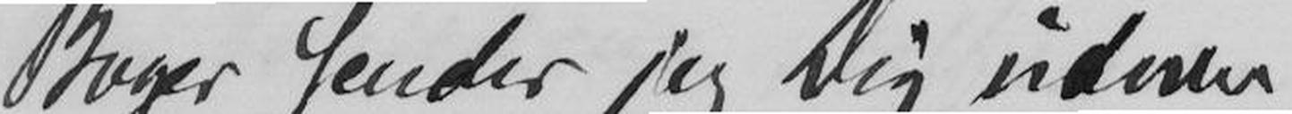Boger sender jeg Dig inden
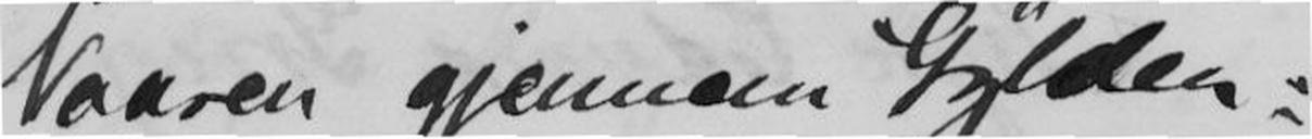Vaaren gjennem Gylden-
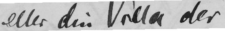eller din Villa der.
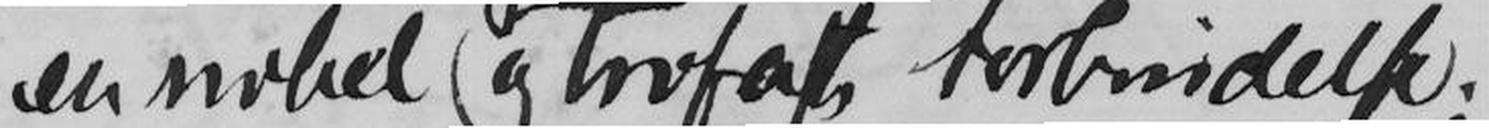en nobel og Trofast Forbindelse;
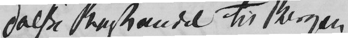dahls Boghandel til Bergen
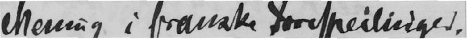Mening i franske Forespeilinger.
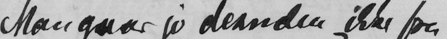Man gaar jo desuden ikke fra
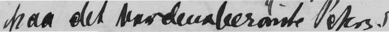paa det værdensberømte Peters
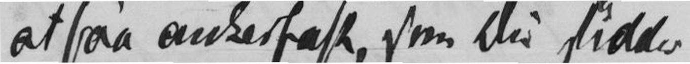at saa ankerfast, som Du sidder
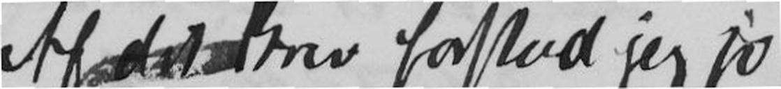Af dit Brev forstod jeg jo,
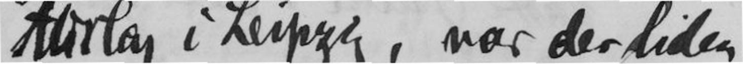Forlag i Leipzig, var der liden
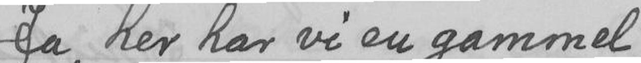Ja, her har vi en gammel
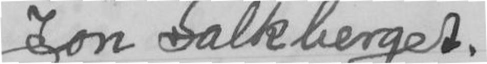Jon Falkberget.
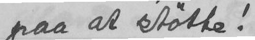paa at støtte!
